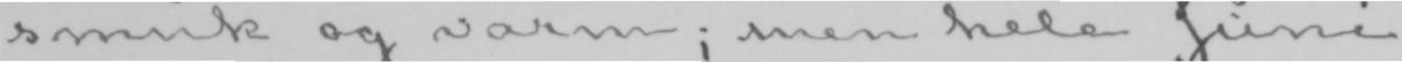smuk og varm; men hele Juni
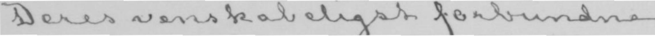Deres venskabeligst forbundne
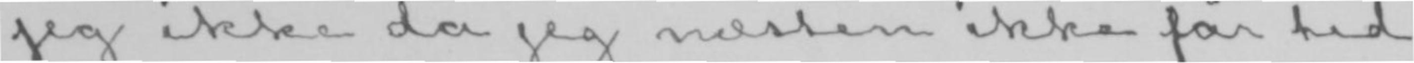jeg ikke da jeg næsten ikke får tid
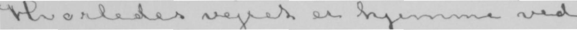Hvorledes vejret er hjemme ved
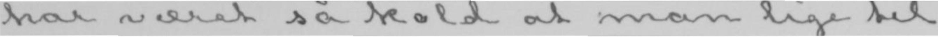har været så kold at man lige til
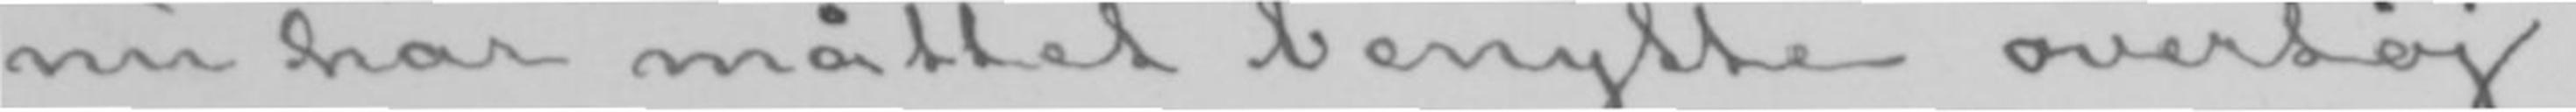nu har måttet benytte overtøj.
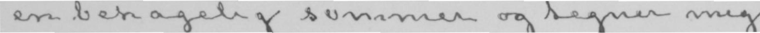en behagelig sommer og tegner mig
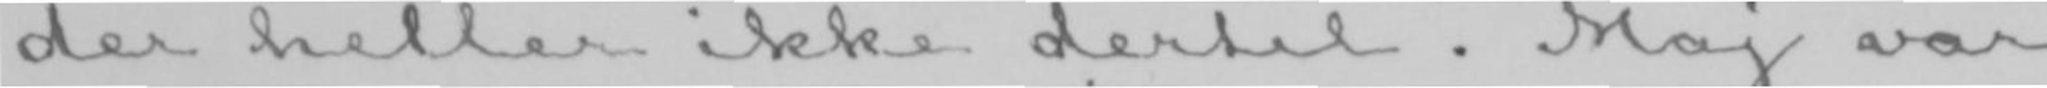der heller ikke dertil. Maj var
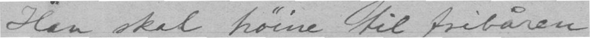Han skal høine til fribåren
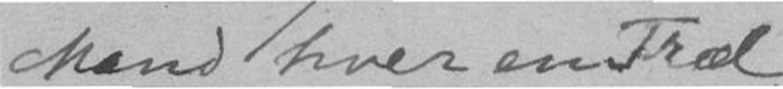Mand hver en træl,
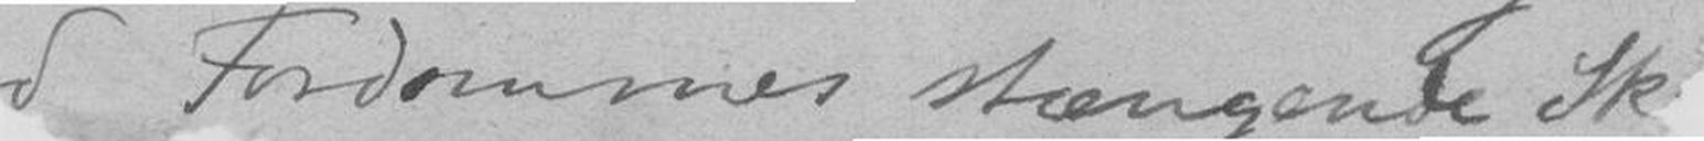Fordommes stængende Sk
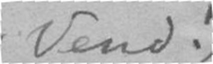(Vend!)
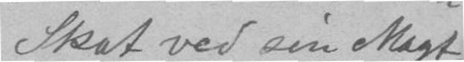Skat ved sin Magt,
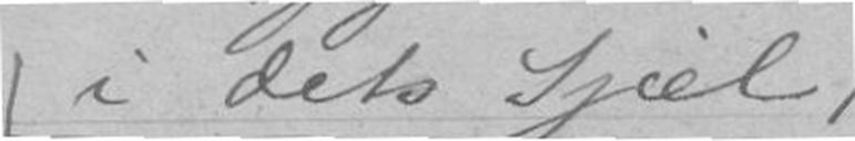i dets Sjæl
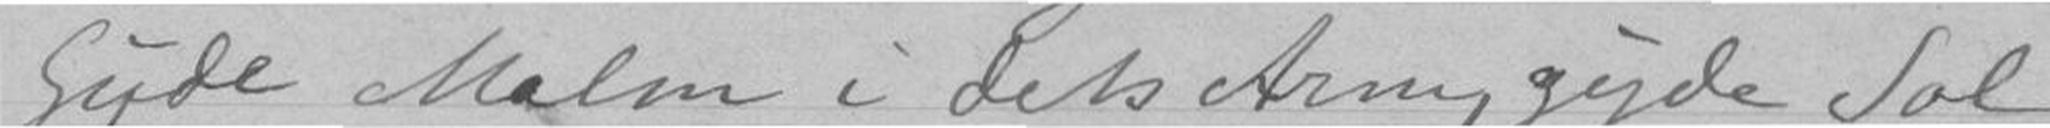Gyde Malm i dets Arm, gyde Sol
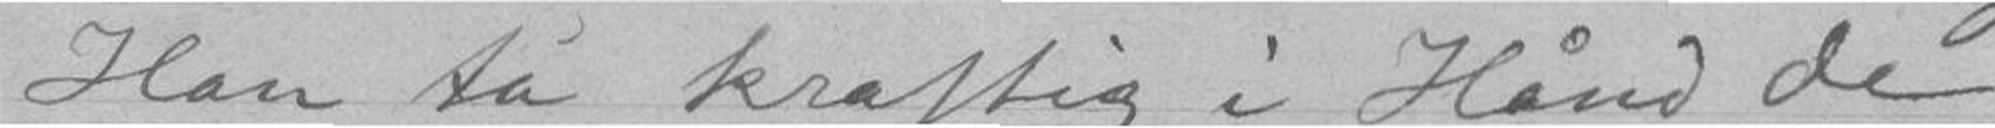Han ta' kraftig i Hånd de
